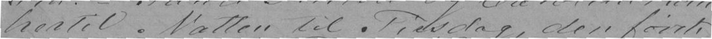hertil Natten til Tirsdag, den første
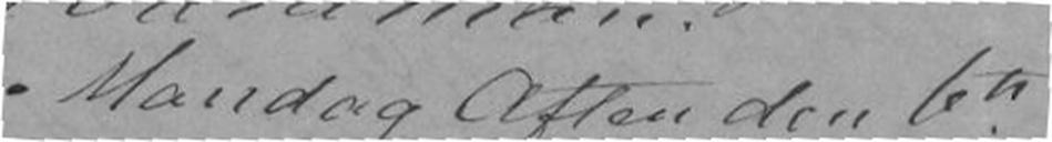Mandag Aften den 6te
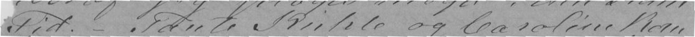Tid. - Tante Kühle og Caroline kom
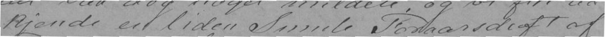kjende en liden Smule Foraarsduft af
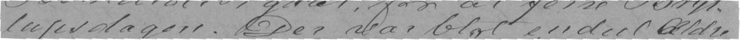lupsdagen. Der var blot endeel Ældre
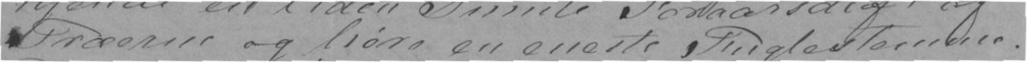Træerne og høre en eneste Fuglestemme.
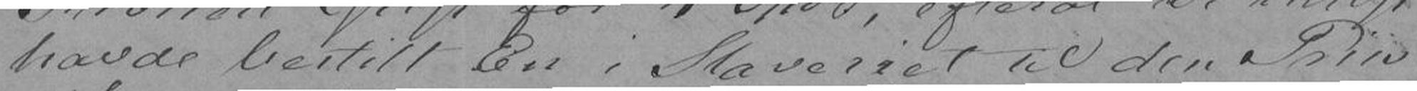havde bestilt En i Slaveriet til den Priis.
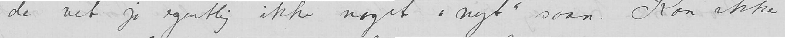de vet jo egentlig ikke noget i «nyt» saan. Kan ikke
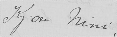Kjære Nini,
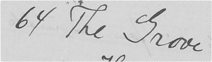64 The Grove
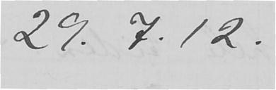29.7.12.
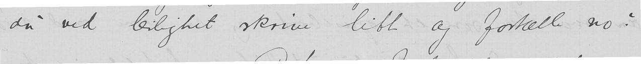du ved leilighet skrive litt og fortælle no?
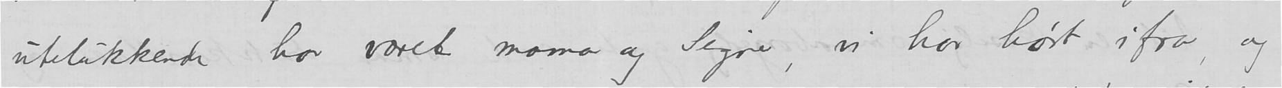utelukkende har været mama og Signe, vi har hørt ifra, og
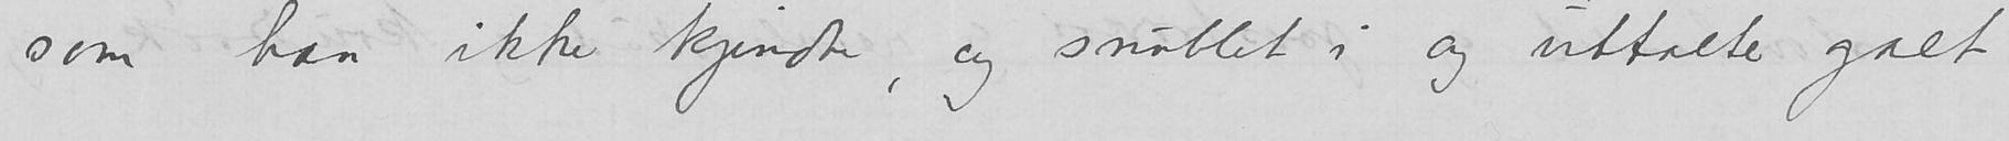som han ikke kjendte, og snublet i og uttalte galt
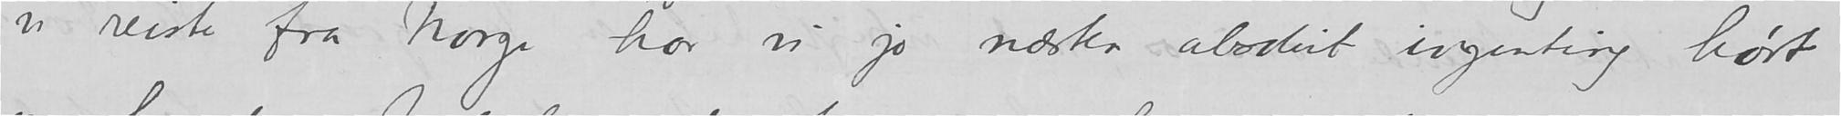vi reiste fra Norge har vi jo næsten alsolut ingenting hørt
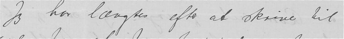Jeg har længtes efter at skrive til
